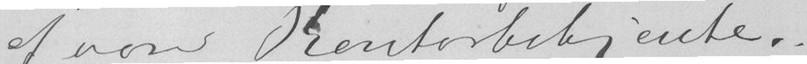af vore Kontorbetjente.
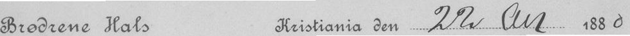Brødrene Hals Kristiania den 22 Oct 1880
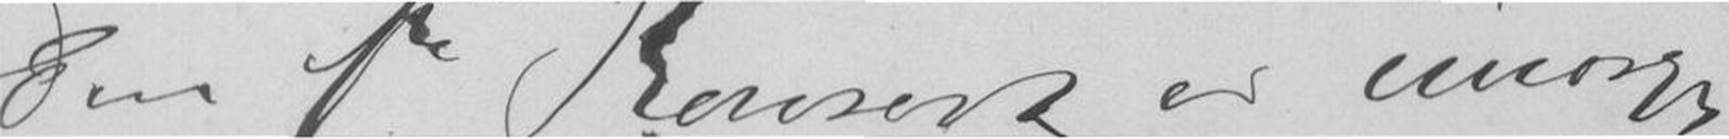Den 1ste Konsert er imorgen
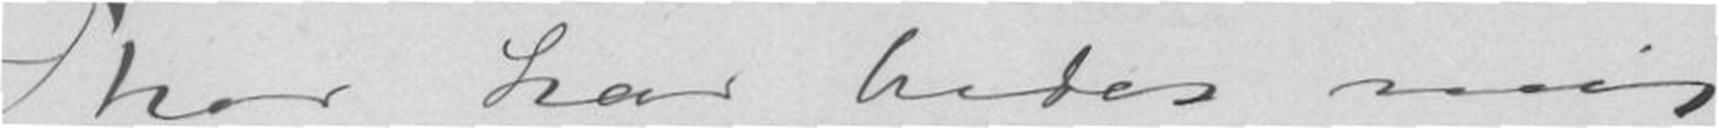Thor har bedet mig
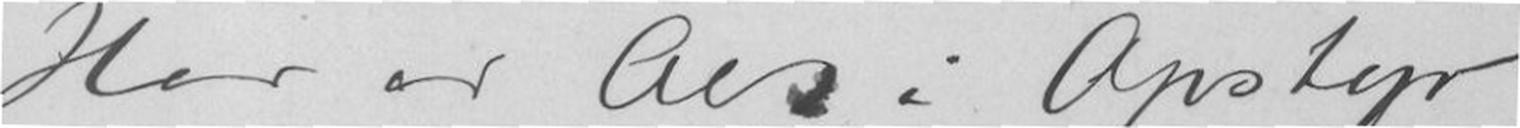Her er Alt i Opstyr
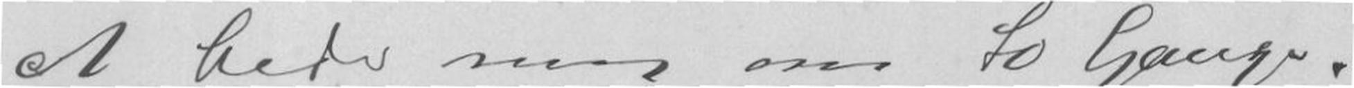at bede mig om to Gange.
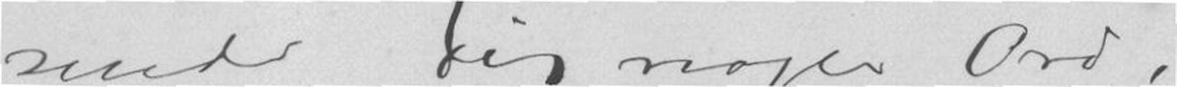sende Dig nogle Ord,
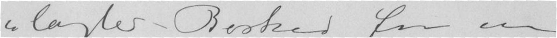lagte Besked fra en
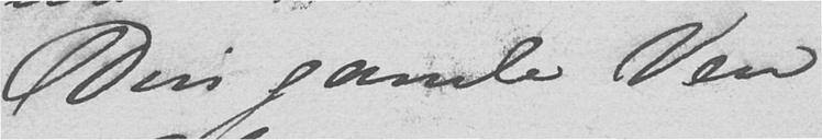Din gamle Ven,
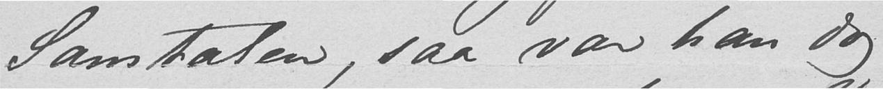Samtalen, saa var han dog
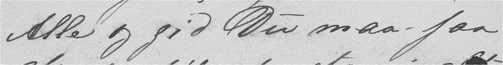Alle og gid Du maa faa
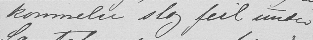kommelse slog feil under
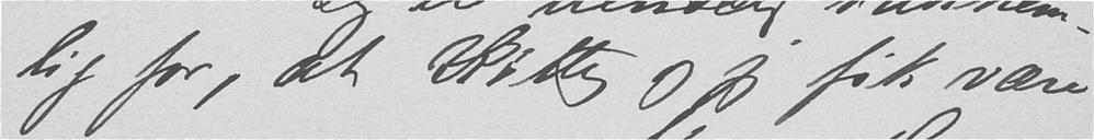lig for, at Kitty og jeg fik være
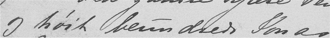og høit beundrede Jonas
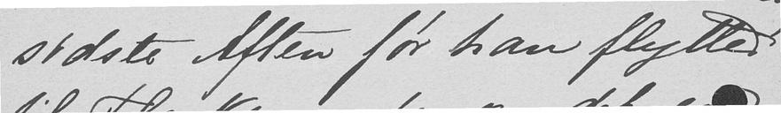sidste Aften før han flytted
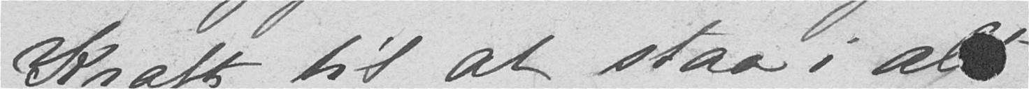Kraft til at staa i alt
